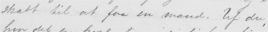skabt til at faa en mand. Uf du,
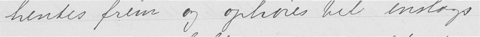hentes frem og ophøies til enslags
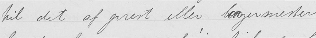til det af prest eller borgermester
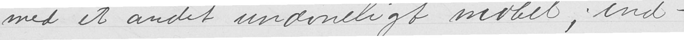med et andet unævneligt møbel; ind-
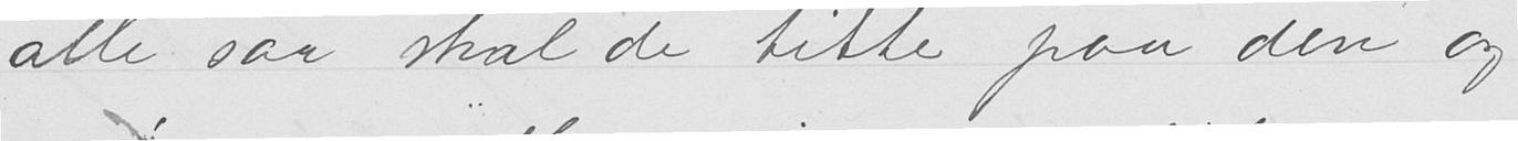alle saa skal de titte paa den og
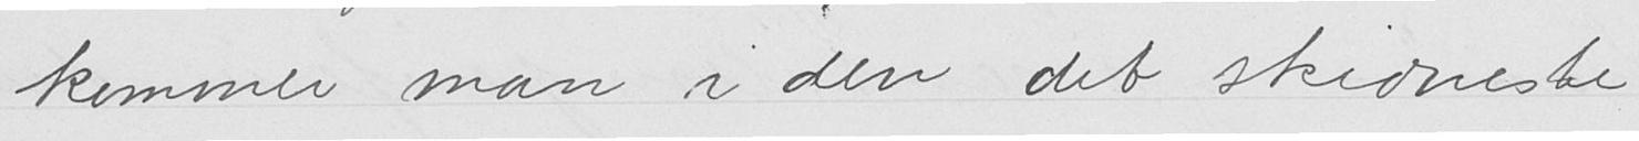kommer man i det den skidneste
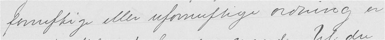fornuftige eller ufornuftige ordning er
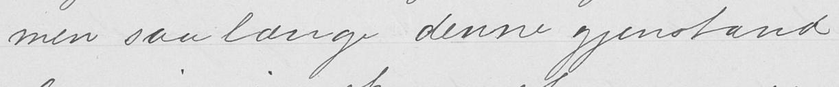men saalænge denne gjenstand
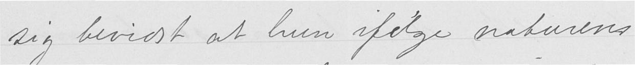sig bevidst at hun ifølge naturens
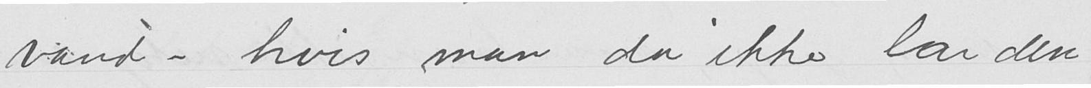vand - hvis man da ikke lar den
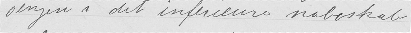sengen i det inferieure naboskab, -
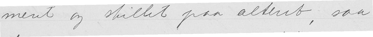meret og stillet paa alteret, saa
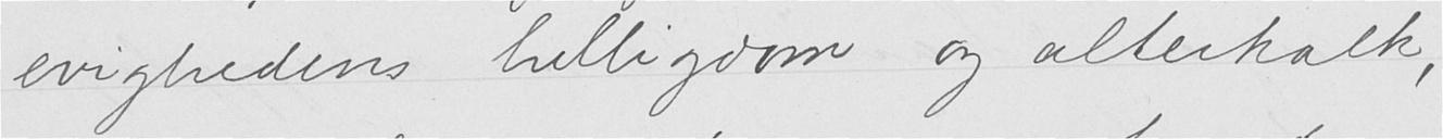evighedens helligdom og alterkalk,
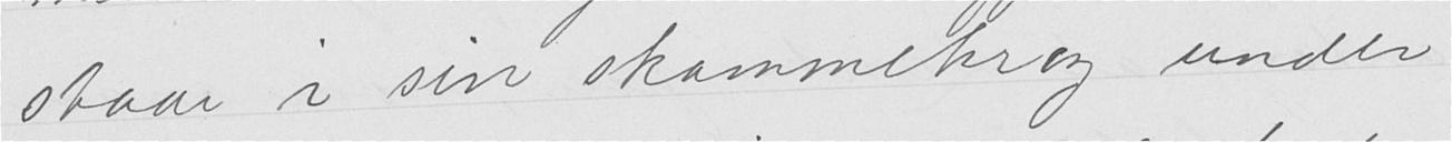staar i sin skammekrog under
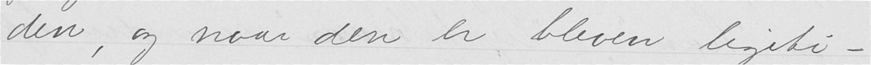den, og naar den er blevet legiti-
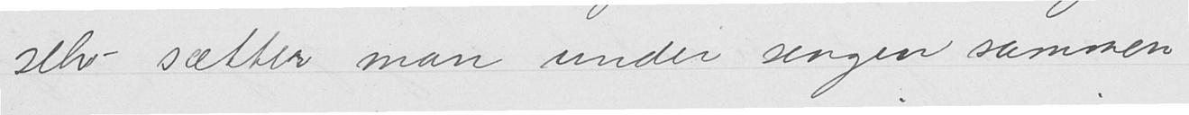selv sætter man under sengen sammen
